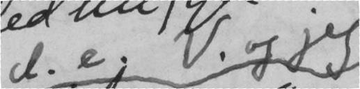d.e V. og jeg
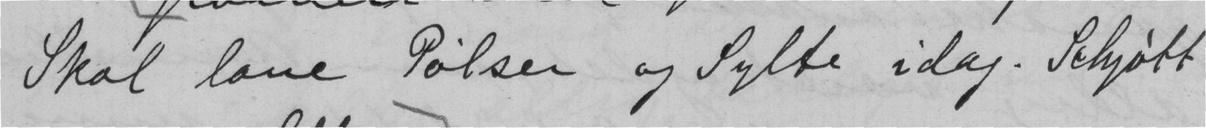Skal lave Pølser og Sylte idag. Schjøtt
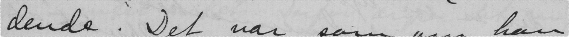dende. Det var som om han
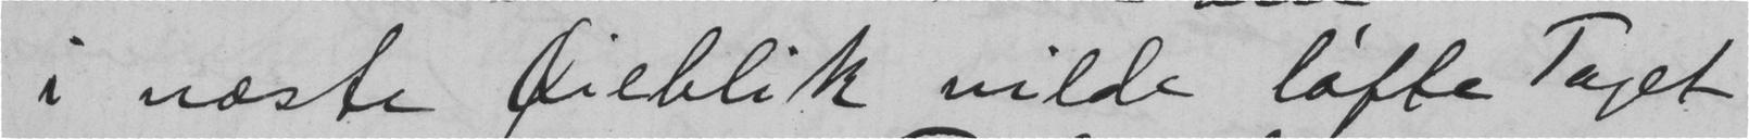i næste Øieblik vilde løfte Taget
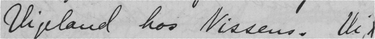Vigeland hos Nissens. Vi,
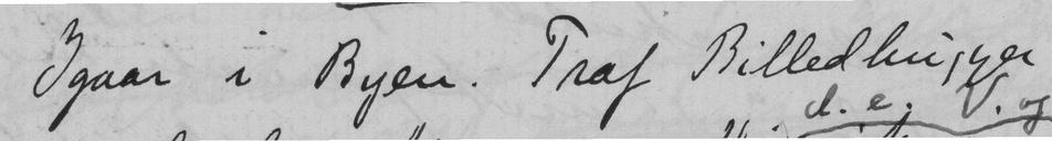Igaar i Byen. Traf Billedhugger
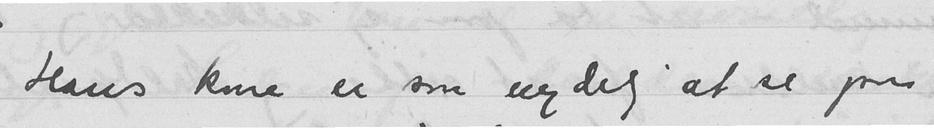Hans kone er saa nydelig at se paa
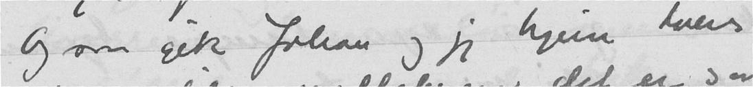Og saa gik Johan og jeg hjem tvers
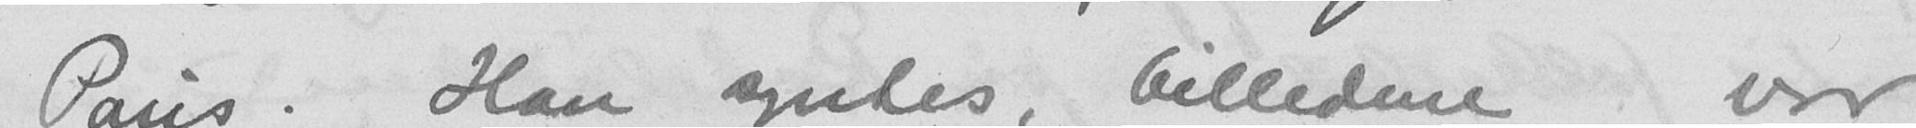Paris. Han syntes, billedene var
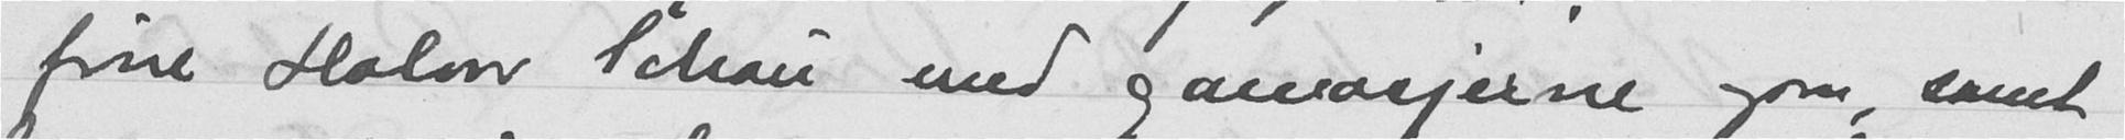fine Halvor Schou med gamasjerne ogsaa, snart
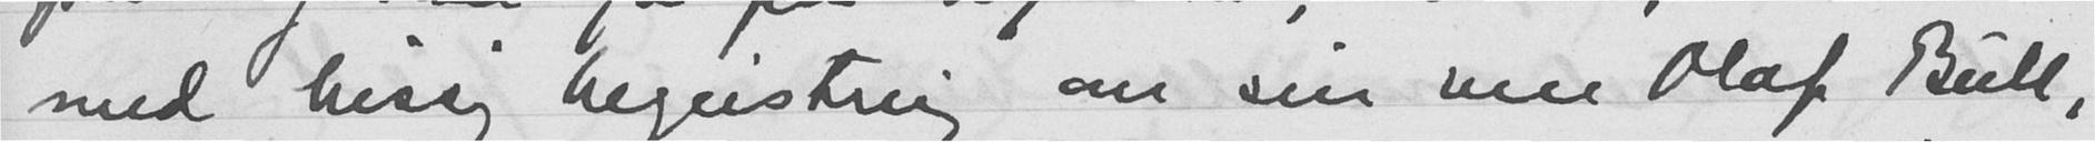med hissig begeistring om sin ven Olaf Bull,
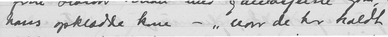hans opklædde kone - "naar de har holdt
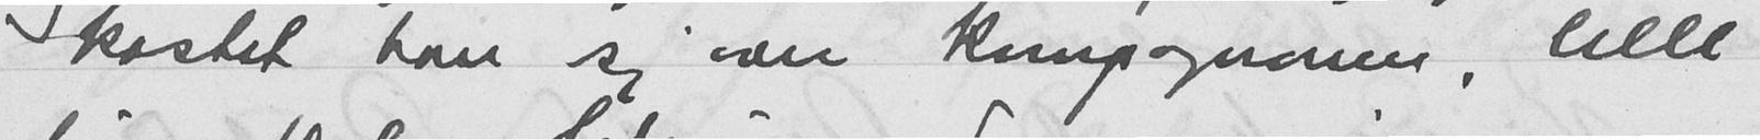kastet han sig over kompagnonen, lille
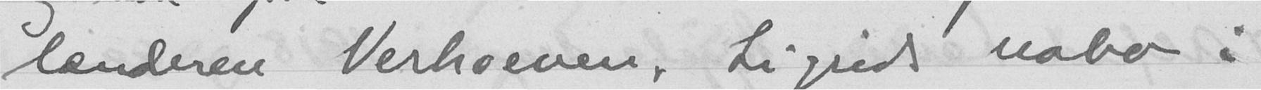lænderen Verhoeven, Sigrids nabo i
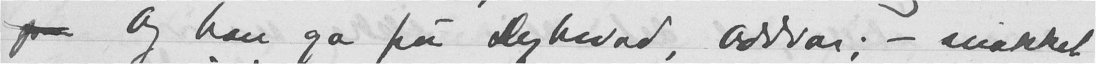paa Og han ga fru Dybwad, Oddvar; - snakket
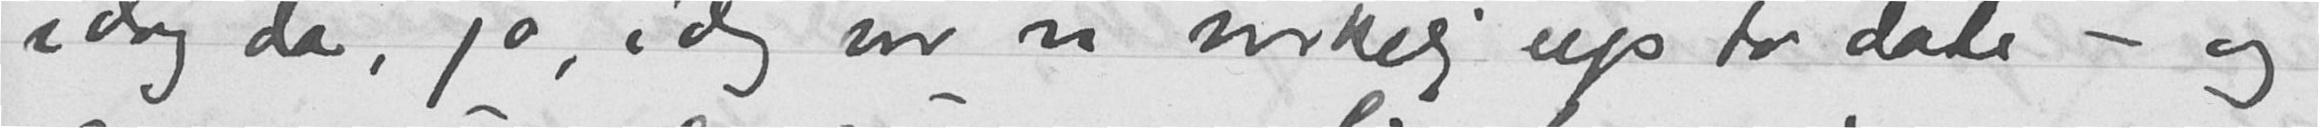i dag da, jo, i dag var vi virkelig up to date - og
